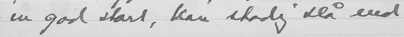en god start, kan stadig stå ved
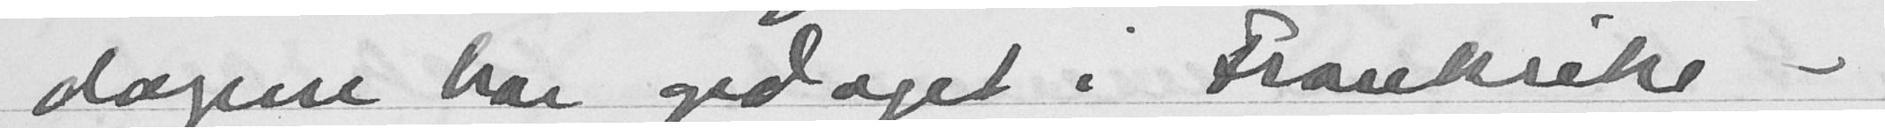dagene har opdaget i Frankrike -
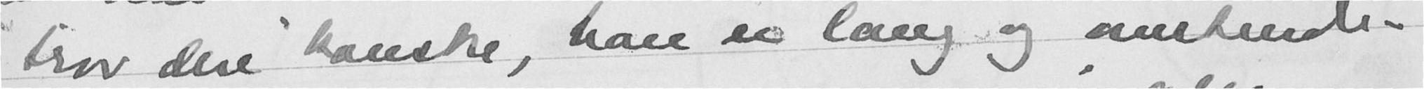tror dere kanskje, han er lang og omstende-
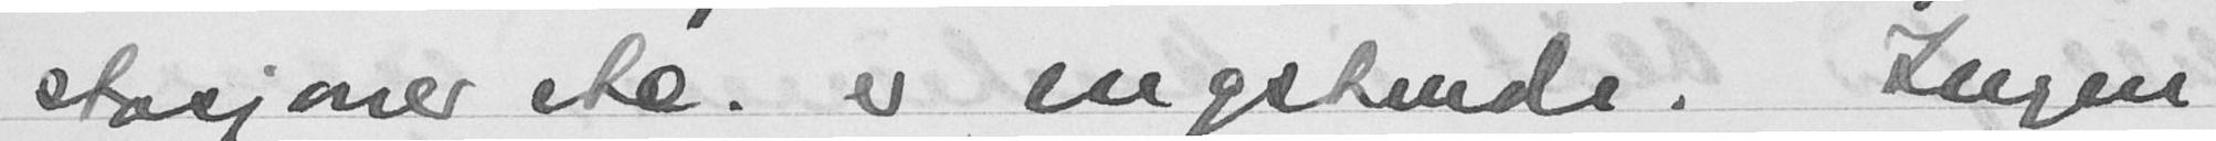stasjoner etc. er engstende. Ingen
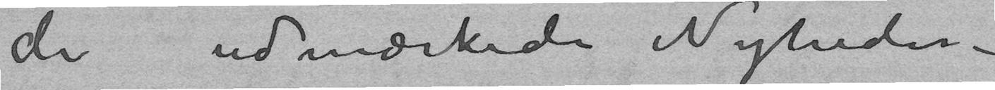de udmærkede Nyheder –
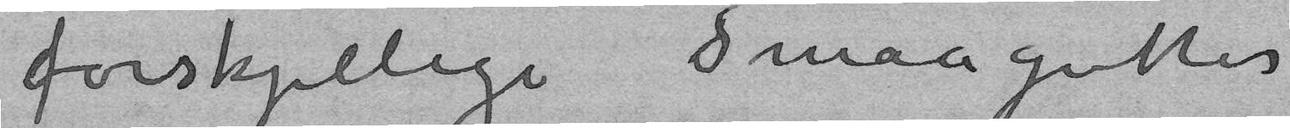forskjellige Smaagutter
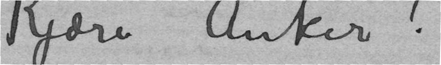Kjære Anker!
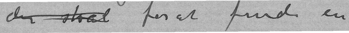der skal forat finde en
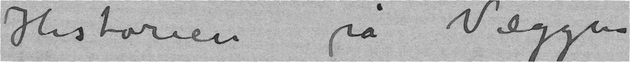Historien på Veggen
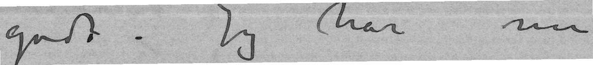godt. Jeg har nu
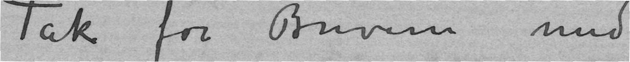Tak for Brevene med
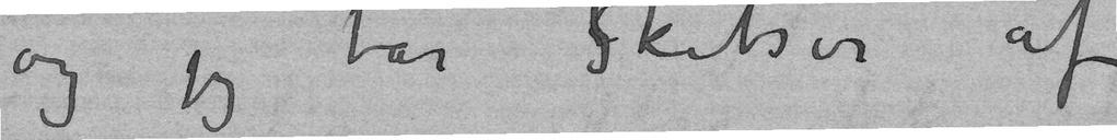og jeg har Skitser af
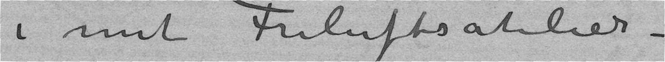i mit Friluftsatelier –
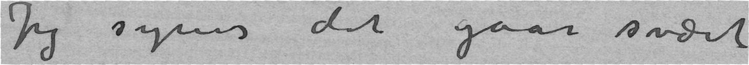Jeg synes det gaar svært
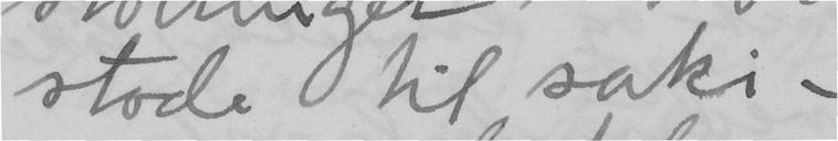stode til saki –
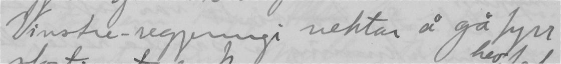Vinstre-regjeringi nektar å gå fyrr
